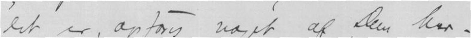det er, opføres noget af Dem her-
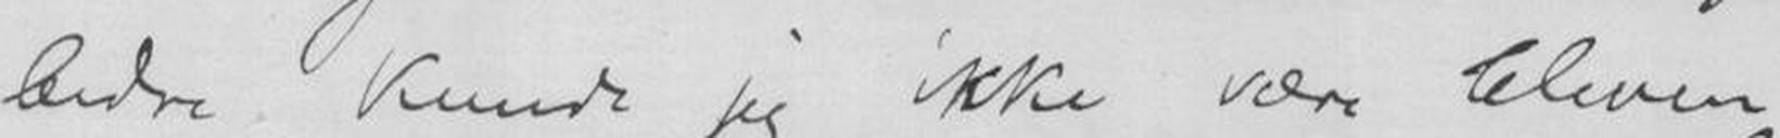bedre kunde jeg ikke være bleven
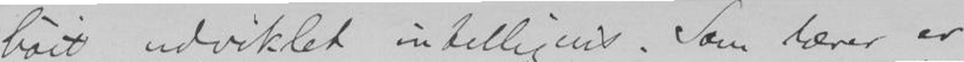høit udviklet intelligens. Som lærer er
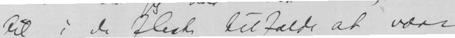til i de fleste tilfælde at være
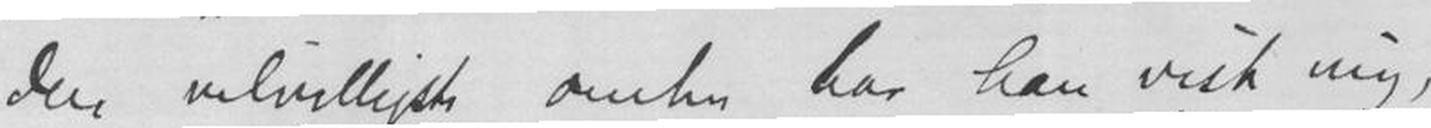den velvilligste omhu har han vist mig,
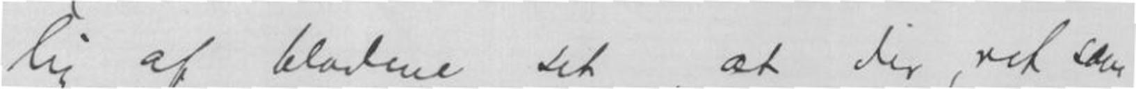lig af bladene set, at der, ret som
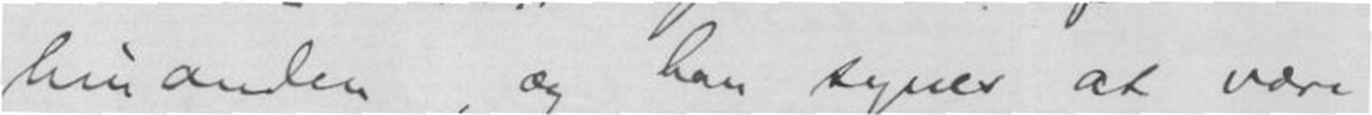hinanden, og han synes at være
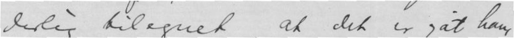derlig tilegnet, at det er gåt ham
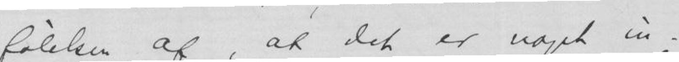følelsen af, at det er noget in-
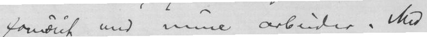fornøiet med mine arbeider. Med
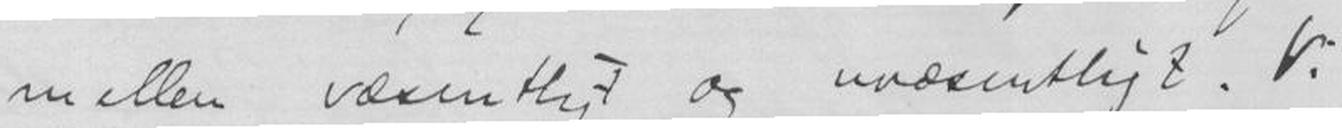mellem væsentligt og uvæsentligt. Vi
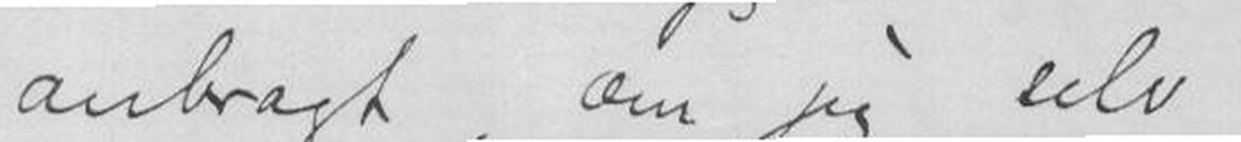anbragt, om jeg selv
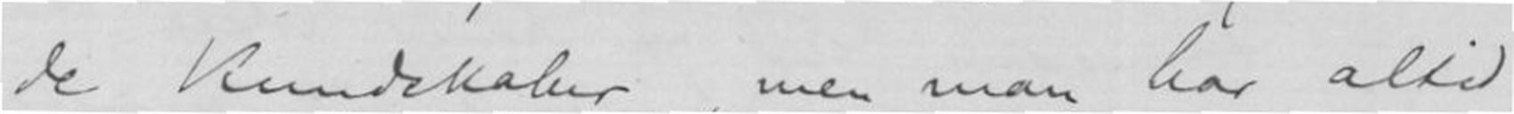kundskaber, men man har altid
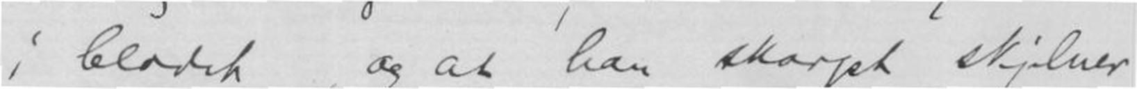i blodet, og at han skarpt skjelner
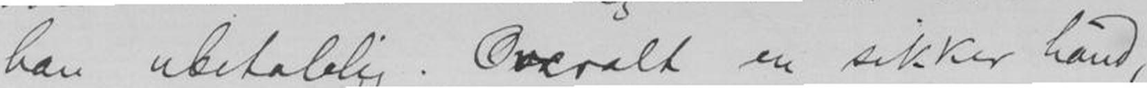han ubetalelig. Overalt en sikker hånd,
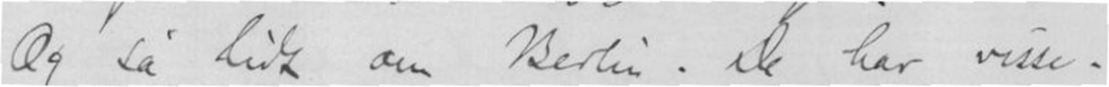Og så lidt om Berlin. De har visse-
